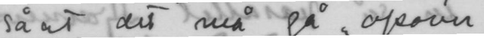så at det må gå opover
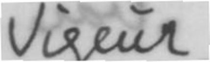Vigeur.
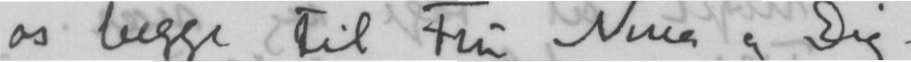os begge til Fru Nina og Dig
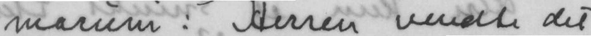marum: Herren vendte det
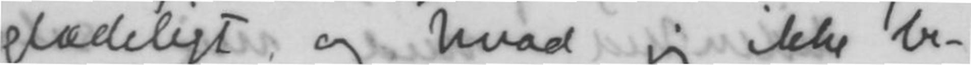Glædeligt og hvad jeg ikke be-
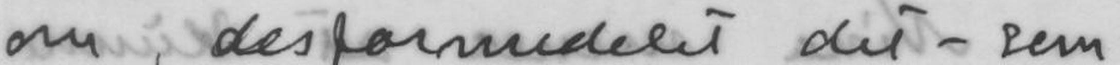om, desformedelet det - som
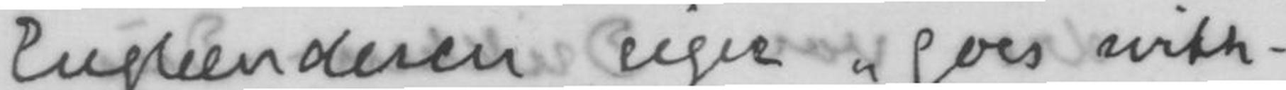Englænderen siger goes with
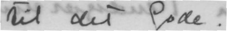til det Gode.
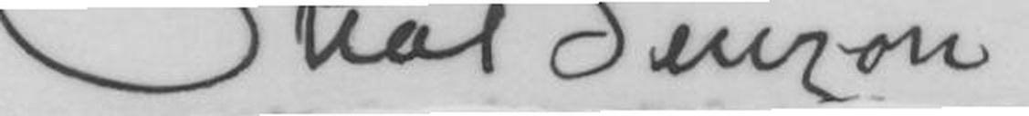Otto Benzon
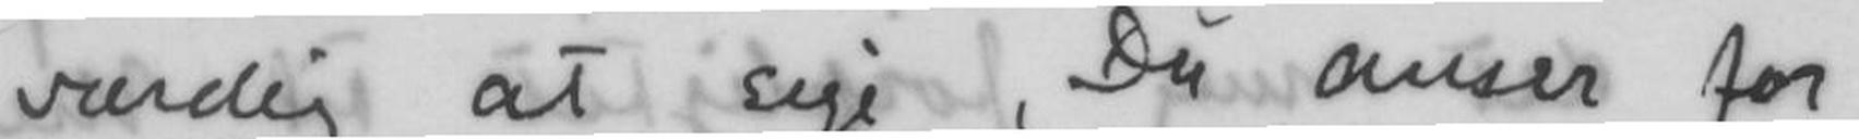værdig at sige, Du anser for
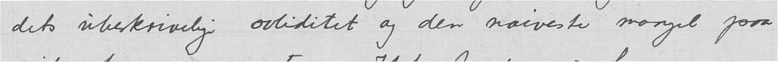dets ubeskrivelige soliditet og den naiveste mangel paa
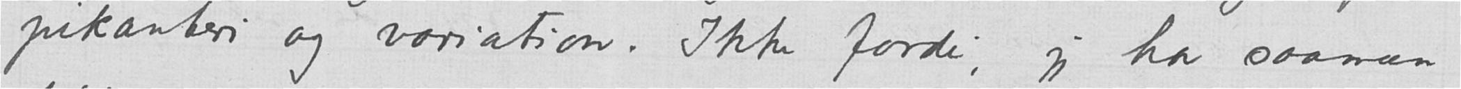pikanteri og variation. Ikke fordi, jeg har saamæn
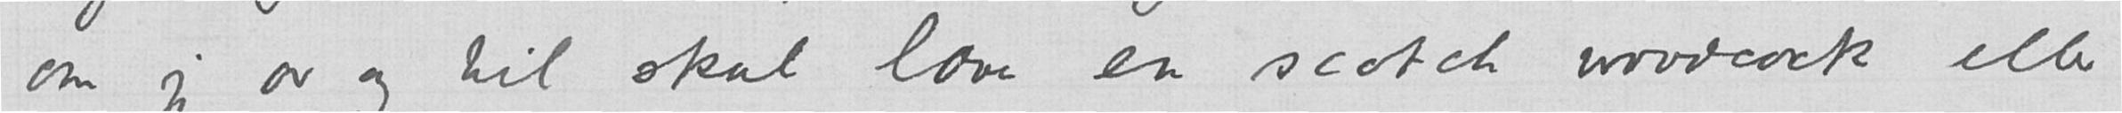om jeg av og til skal lave en scotch woodcock eller
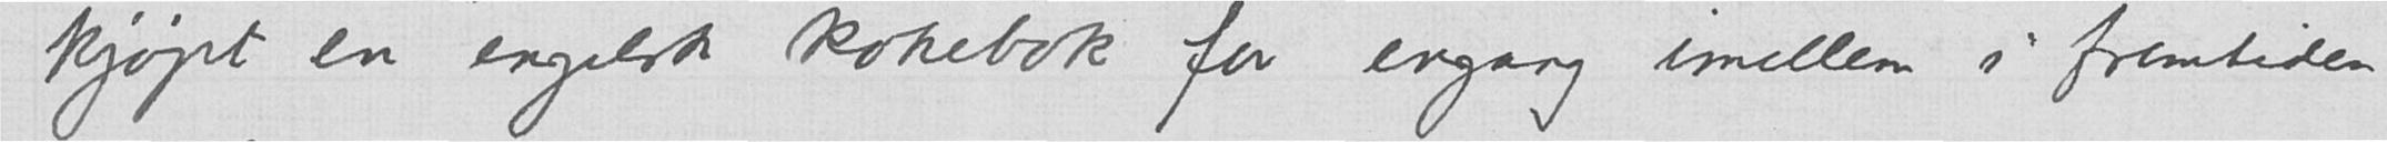kjøpt en engelsk kokebok for engang imellem i fremtiden
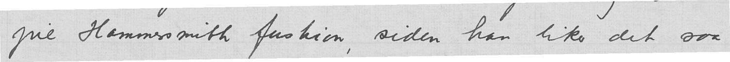pie Hammersmith fashion, siden han liker det saa
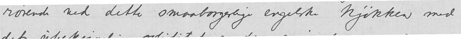rørende ved dette smaaborgerlige engelske kjøkken med
